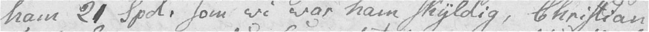ham 21 Spd, som vi var ham skyldig, Christian
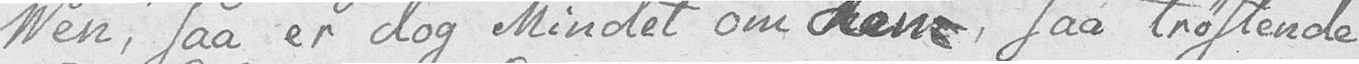Ven, saa er dog Mindet om dem, saa trøstende
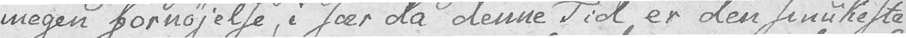megen fornøjelse, i sær da denne Tid er den smukeste
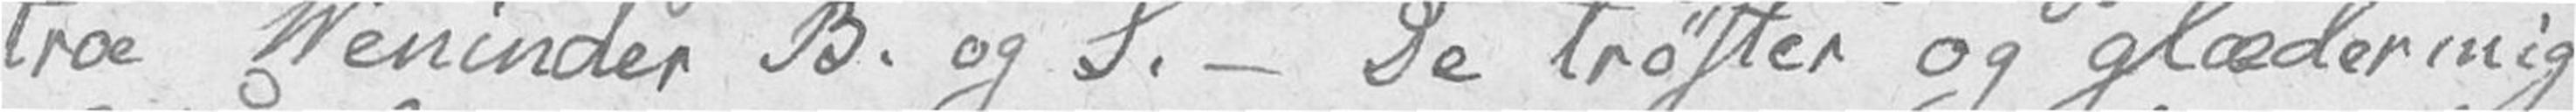troe Veninder B. og S. – De trøster og glæder mig
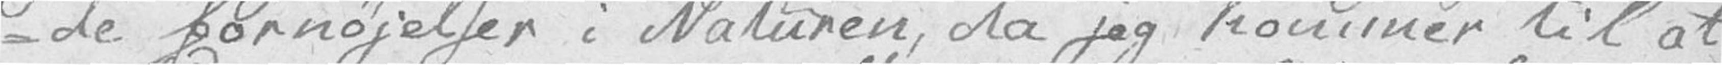-de fornøjelser i Naturen, da jeg kommer til at
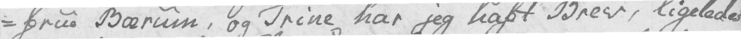-frue Bærum, og Trine har jeg haft Brev, ligeledes
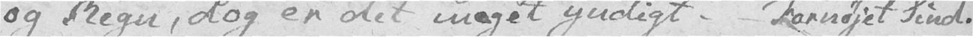og Regn, dog er det meget yndigt. – Fornøjet Sind.
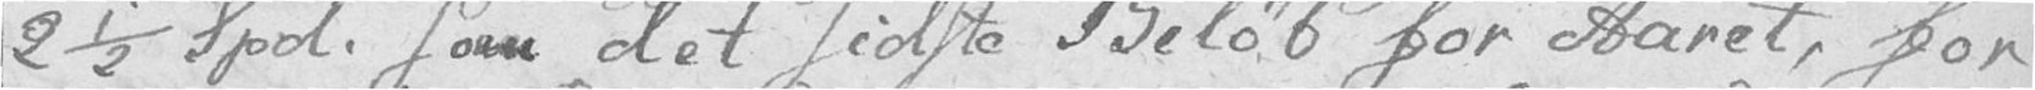2 ½ Spd. som det sidste Beløb for Aaret, for
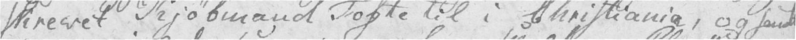skrevet Kjøbmand Tofte til i Christiania, og sendt
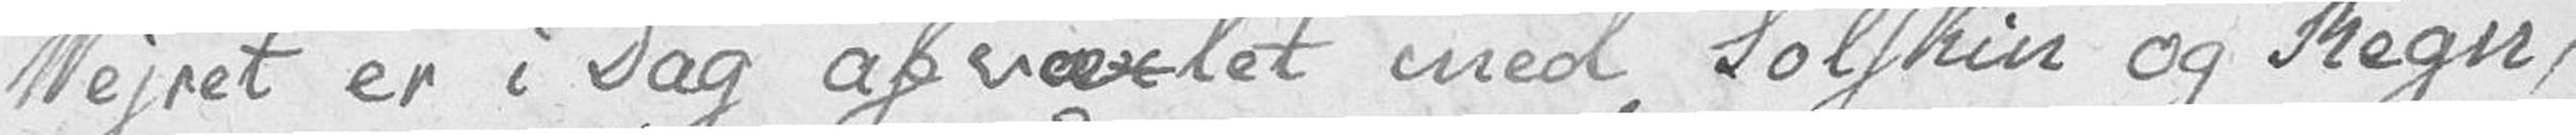Vejret er i Dag afvæxlet med Solskin og Regn,
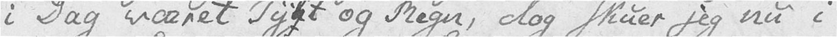i Dag været Tykt og Regn, dog skuer jeg nu i
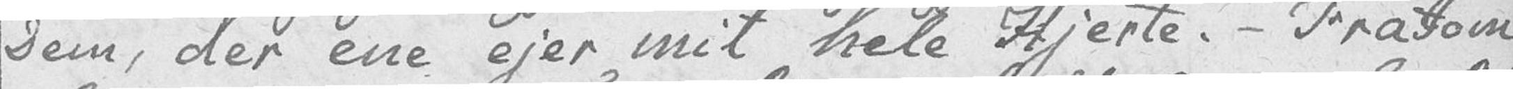Dem, der ene ejer mit hele Hjerte. – Fra Jom
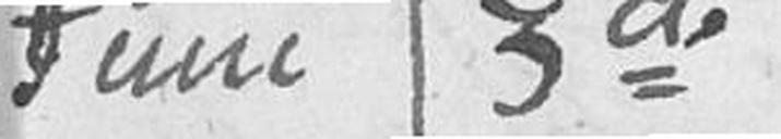Juni 3de
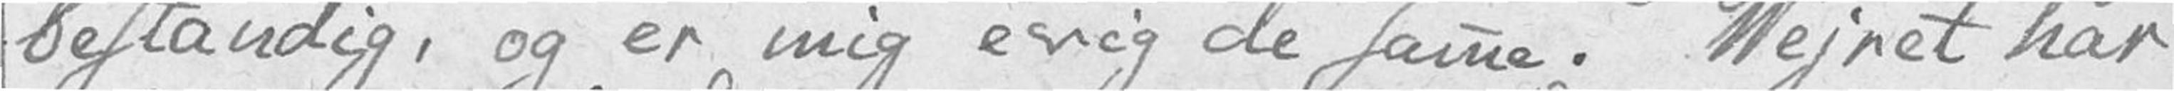bestandig, og er mig evig de samme. Vejret har
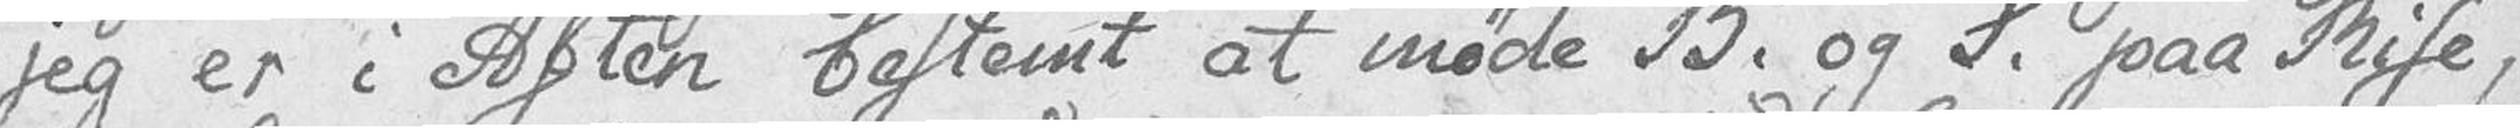jeg er i Aften bestemt at møde B. og S. paa Rife,
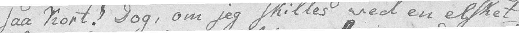saa Kort! Dog, om jeg skilles ved en elsket
